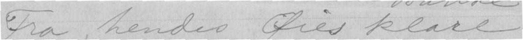Fra, hendes Øies klare
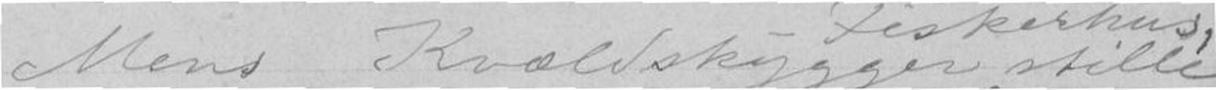Mens Kvældenskygger stille
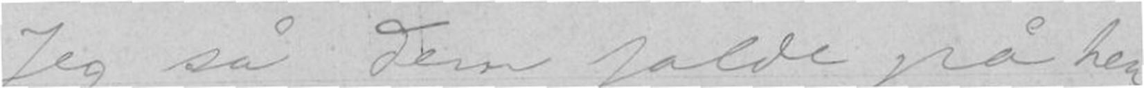Jeg så dem falde på hen-
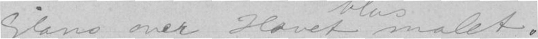Glans over Hovet malet.
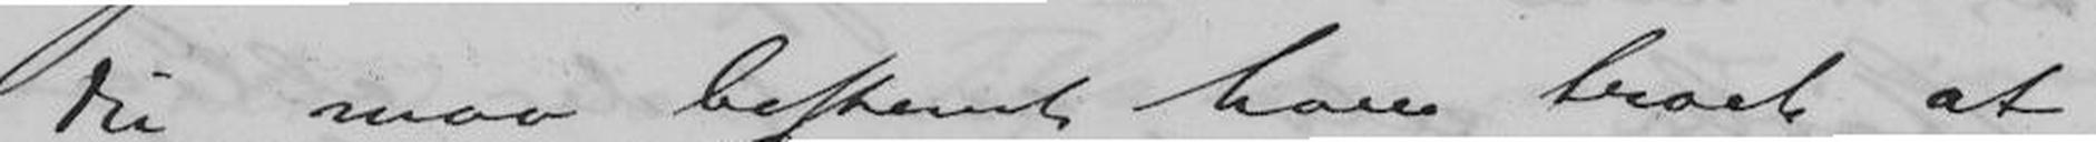Du maa bestemt have troet at
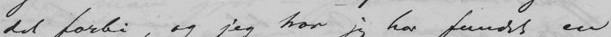det forbi, og jeg tror jeg har fundet en
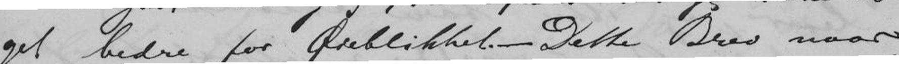get bedre for Øieblikket. - Dette Brev naar
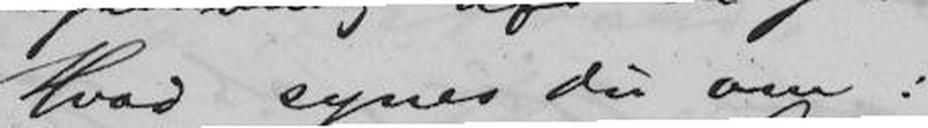Hvad synes du om:
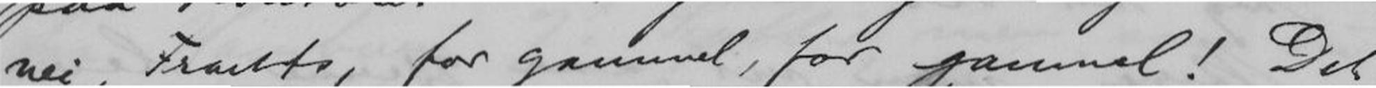nei, Frants, for gammel, for gammel! Det
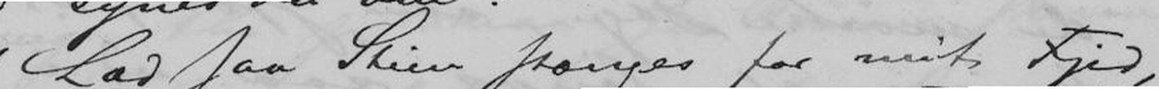"Lad saa Stien stanges for mit Fjed,
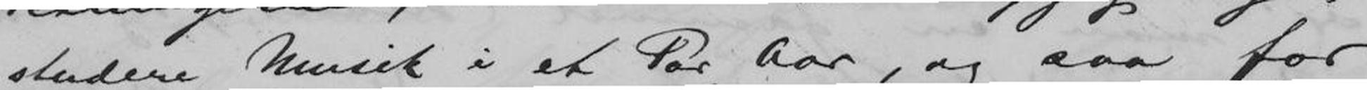studere Musik i et Par Aar, og saa for
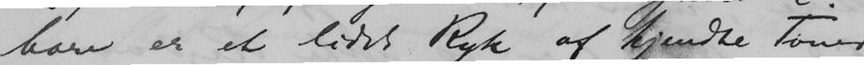bare er et lidet Ryk af kjendte Toner
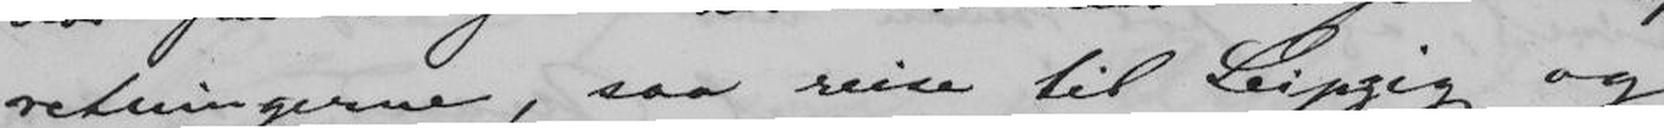retningerne, saa reise til Leipzig og
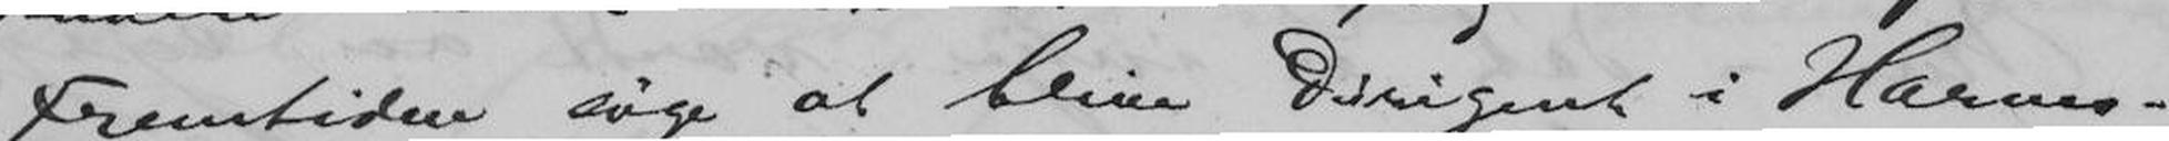fremtiden søge at blive Dirigent i Harmo-
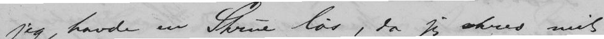jeg havde en Skrue løs, da jeg skrev mit
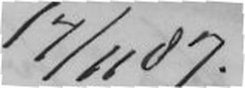17/11 87
